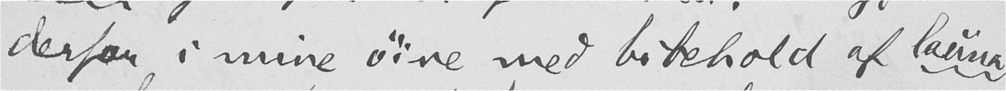derfor i mine øine med bibehold af launa
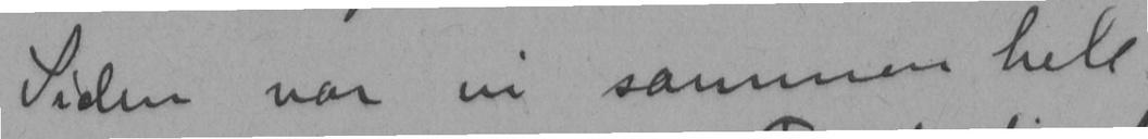Siden var vi sammen hele
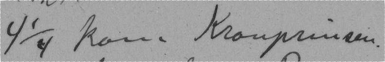4 1/4 kom Kronprinsen.
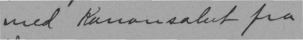med Kanonsalut fra
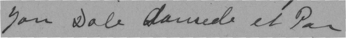Jon Dale dansede et Par
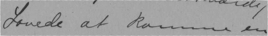Lovede at komme en
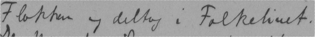Flokken og deltog i Folkelivet.
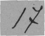17
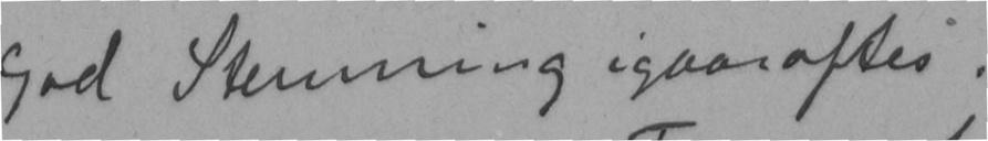God Stemning igaaraftes.
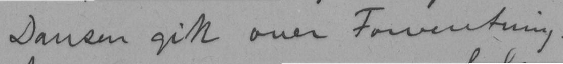Dansen gik over Forventning.
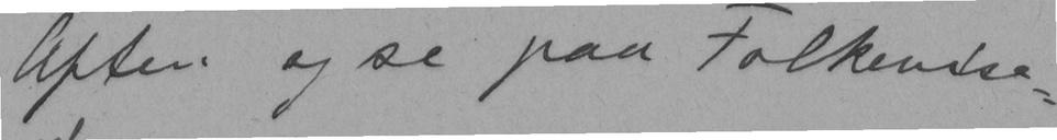Aften. og se paa Folkevise-
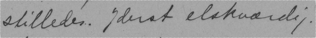stilledes. Iderst elskværdig.
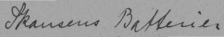Skansens Batterier
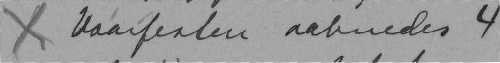x Vaarfesten aabnedes 4
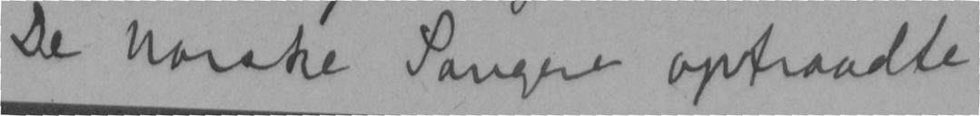De norske Sangere optraadte
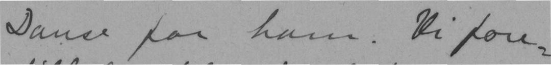Danse for ham. Vi fore-
